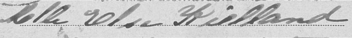lille Else Kielland
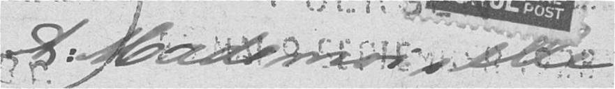A: Mademoiselle
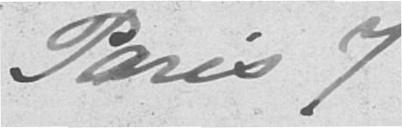Paris 7
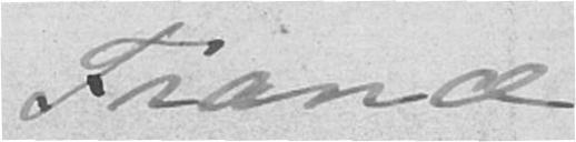France
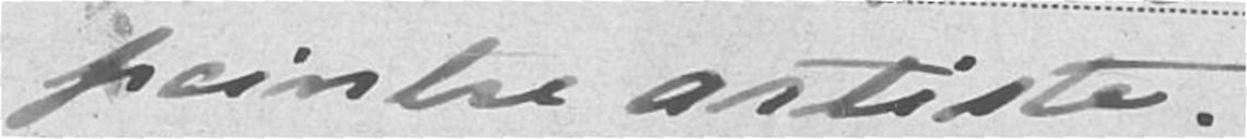paintre artiste.
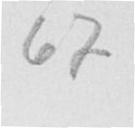67.
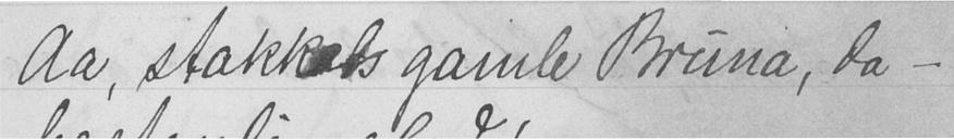Aa, stakkels gamle Bruna, da -
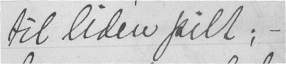til liden pilt; -
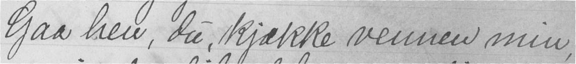Gaa hen, du, kjække vennen min,
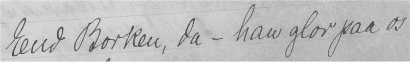End Borken, da - han glor paa os
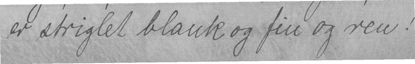er striglet blank og fin og ren!
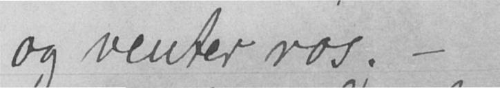og venter ros; -
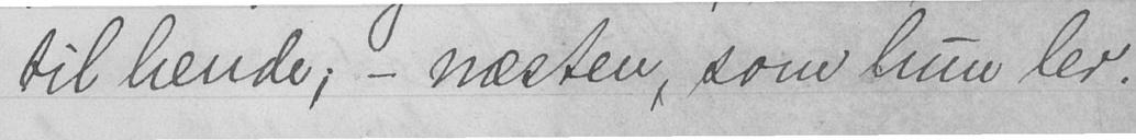til hende; - næsten, som hun ler.
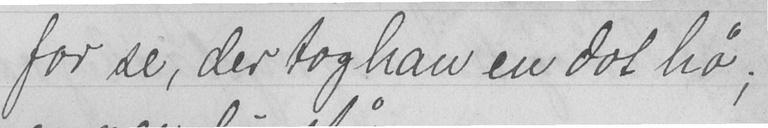for se, der tog han en dot hø;
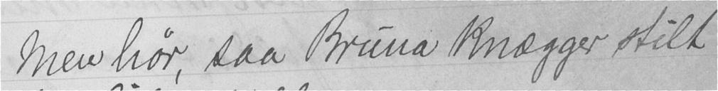Men hør, saa Bruna knægger stilt
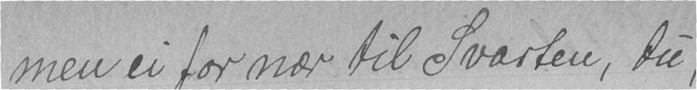men ei for nær til Svarten, du, -
